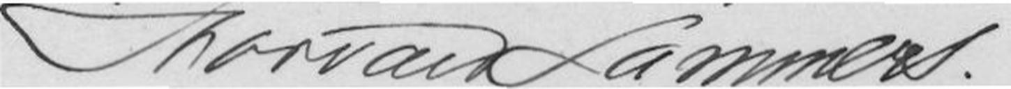Thorvald Lammers
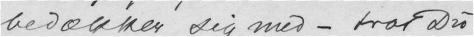bedækker sig med- tror Du
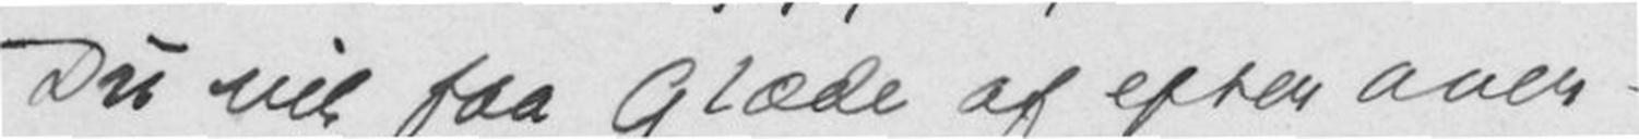Du vil faa Glæde af efter over-
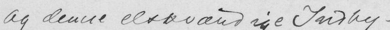og denne elskværdige Indby-
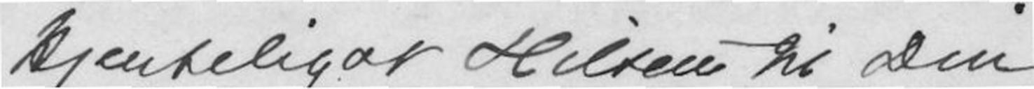Hjerteligst Hilsen til din
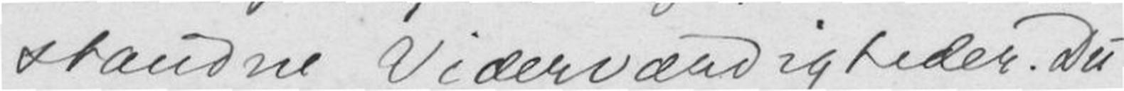standne Videreværdigheder. Du
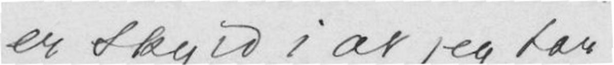er Skyld i at jeg har
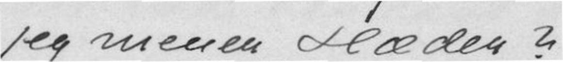jeg mener slæden?
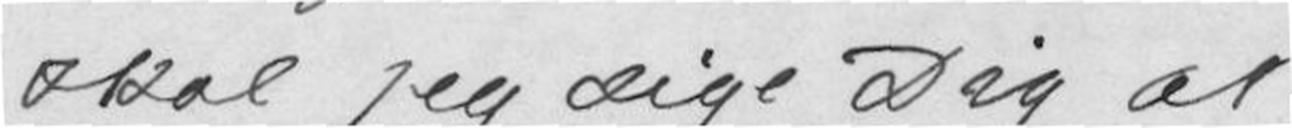skal jeg sige Dig at
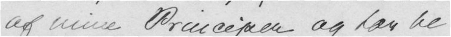af mine og har be
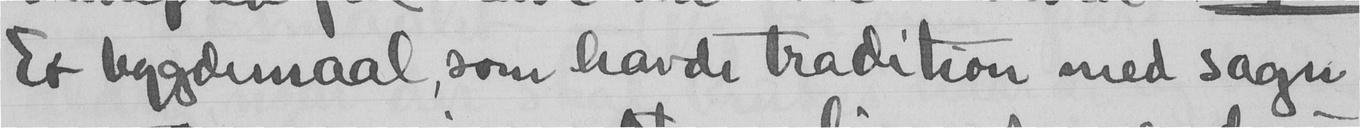Er bygdemaal, som havde tradition med sagn
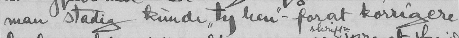man stadig kunde "ty hen" - for at korrigere
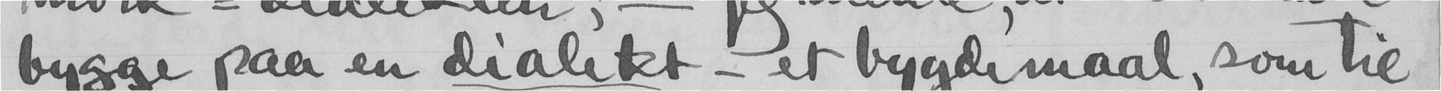bygge paa en dialekt - et bygdemaal, som til
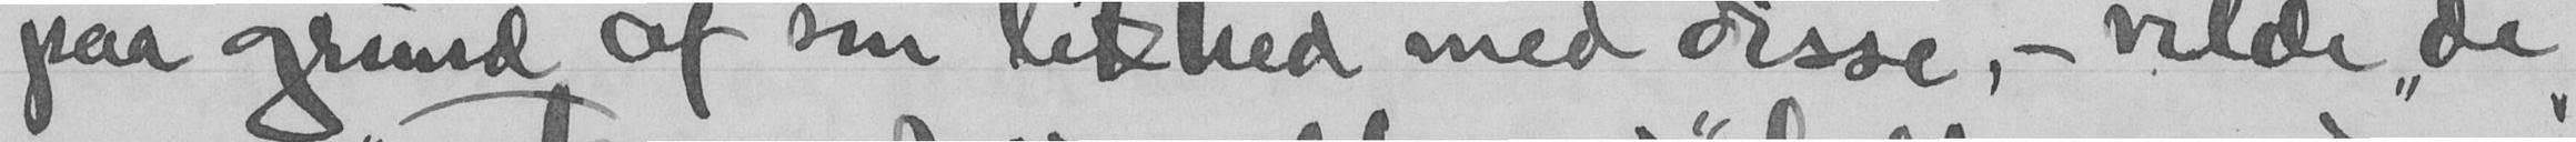paa grund af sin likhed med disse, - vilde "de
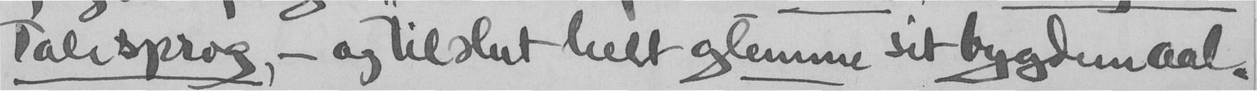talesprog, - og tilslut helt glemme sit bygdemaal.
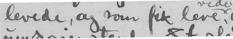levede, og som fik leve,
